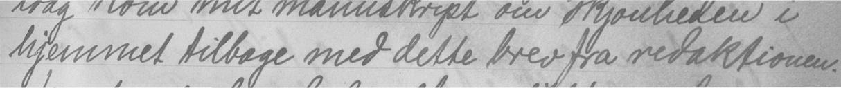hjemmet tilbage med dette brev fra redaktionen.
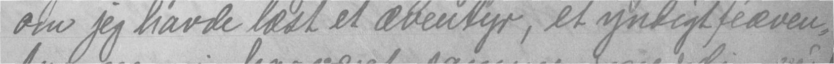om jeg havde læst et æventyr, et yndigt fe'æven-
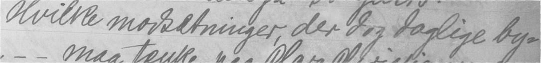Hvilke modsætninger der dog daglige by-
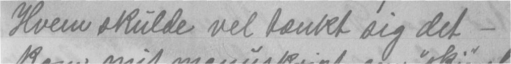Hvem skulde vel tænkt sig det -
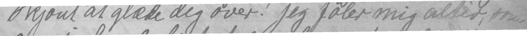skjønt at glæde dig over! jeg føler mig altid, som
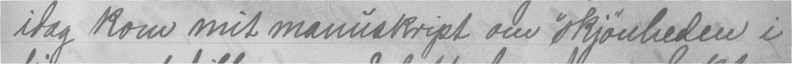idag kom mit manuskript om "skjønheden i
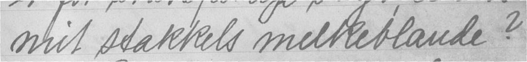mit stakkels melkeblande?
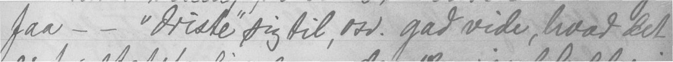faa - - "driste" sig til, osv. gad vide, hvad det
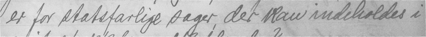er for statsfarlige sager, der kan indeholdes i
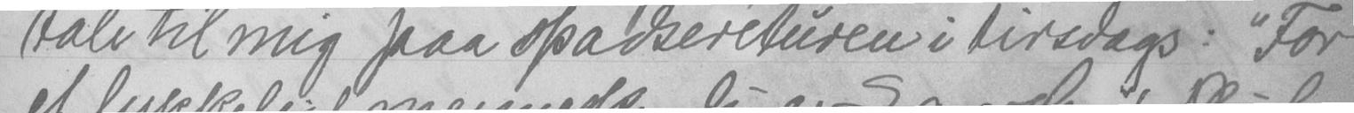tale til mig paa spadsereturen i tirsdags: "For
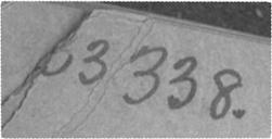338.
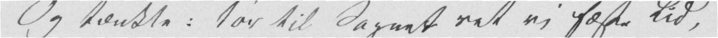Og tænkte: tør til Sagnet ret vi fæste Lid,
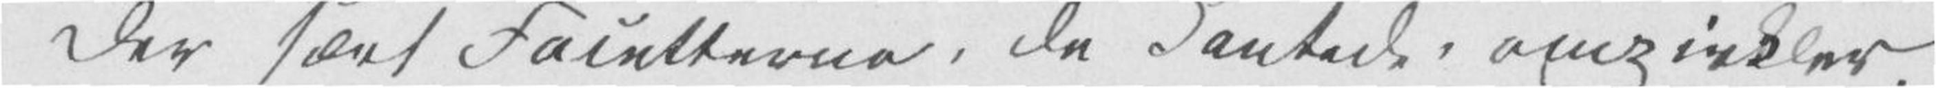Der sært Facetterne, de kantede omzirkler,
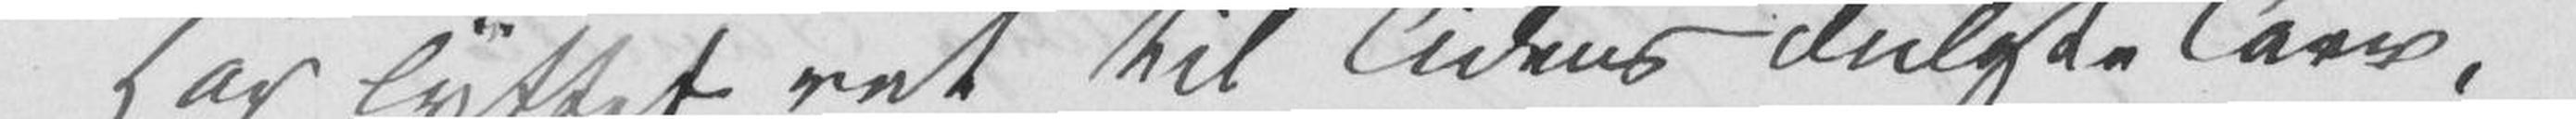Har lyttet ret til Tidens dulgte Tarv,
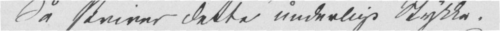Så skriver dette underlige Stykke
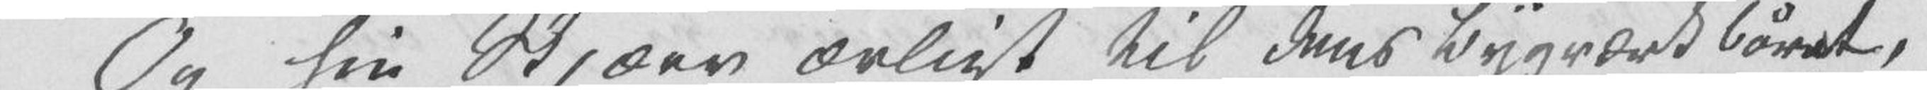Og sin Skjærv ærligt til dens Bygværk båret,
